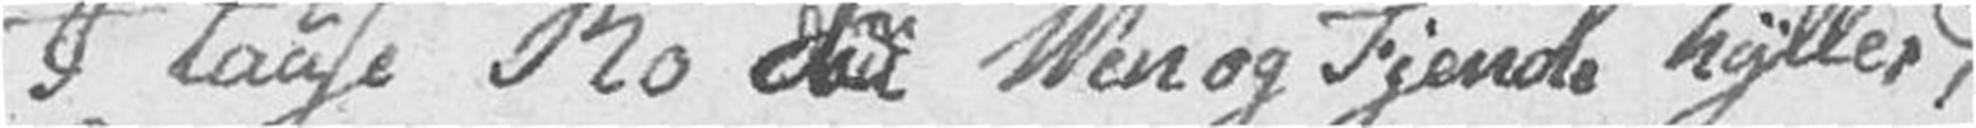I tause Ro du Ven og Fjende hyller,
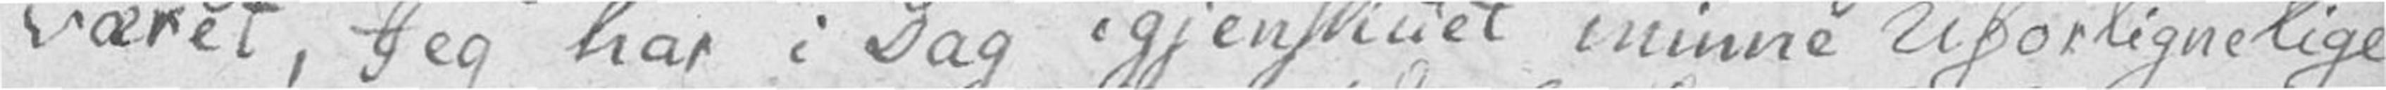været, Jeg har i Dag igjen skuet minne Uforlignelige
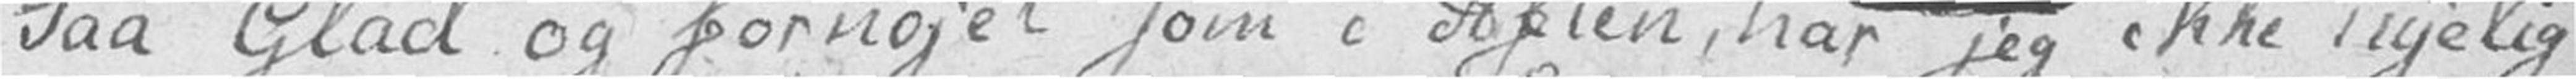Saa Glad og fornøjet som i Aften, har jeg ikke nyelig
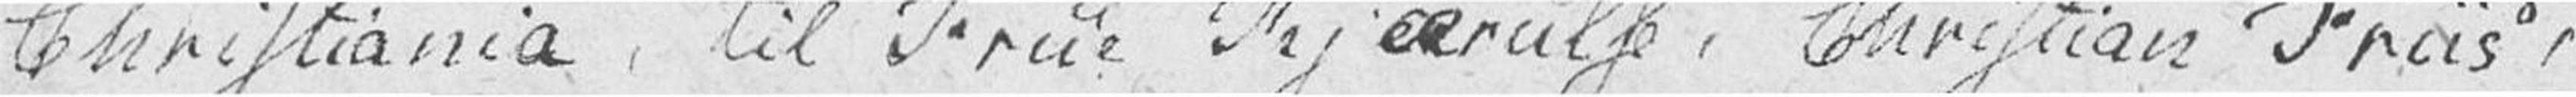Christiania, til Frue Kjærulf, Christian Friis,
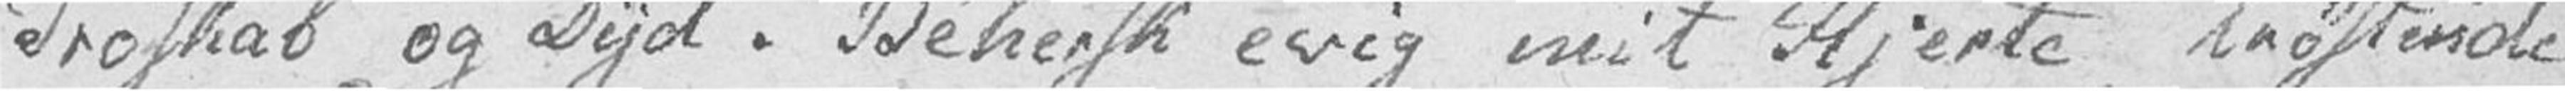Troskab og Dyd. Behersk evig mit Hjerte trøstende
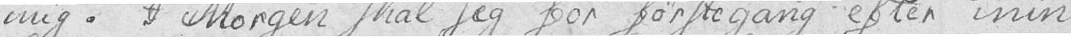mig. I Morgen skal jeg for første gang efter min
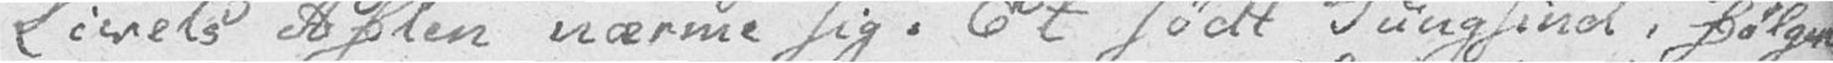Livets Aften nærme sig. Et sødt Tungsind, følger
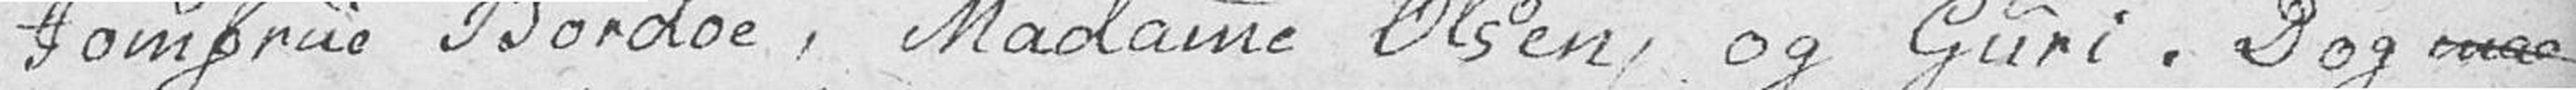Jomfrue Bordoe, Madamme Olsen, og Guri. Dog maa
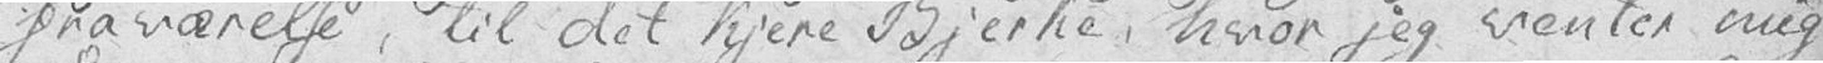fraværelse, til det kjere Bjerke, hvor jeg venter mig
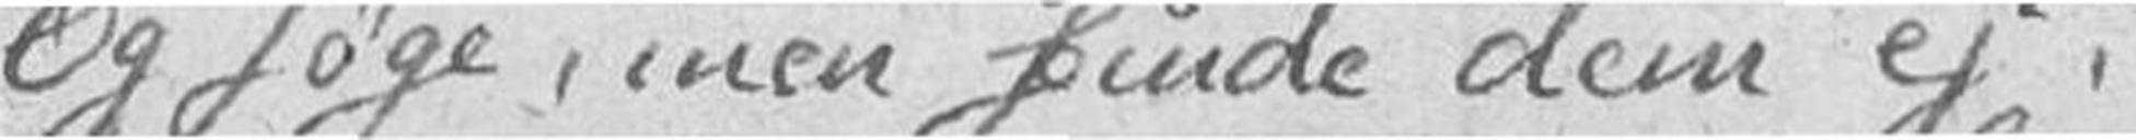Og søge, men finde dem ej.
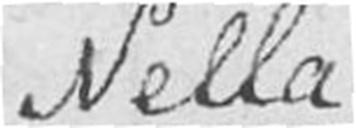Nella
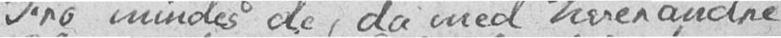Fro mindes de, da med hverandre
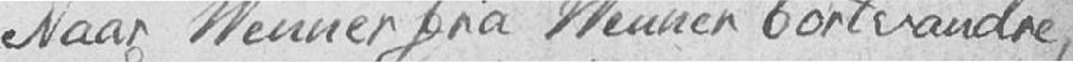Naar Venner fra Venner bortvandre,
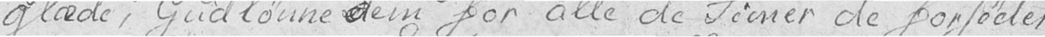glæde, Gud lønne dem for alle de Timer de forsøder
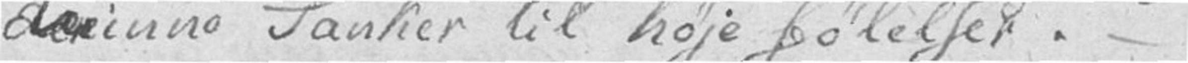minne Tanker til høje følelser. –
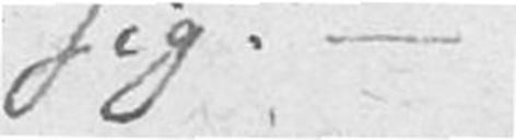sig. –
